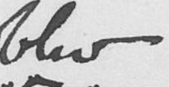blev
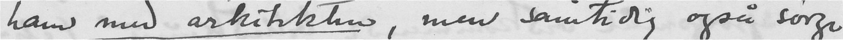ham med arkitekten, men samtidig også sørge
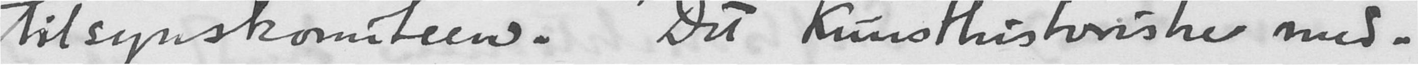Tilsynskomiteen. "Det kunsthistoriske med-
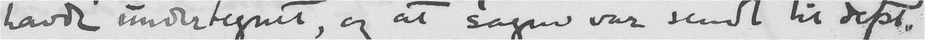havde undertegnet, og at sagen var sendt til dept.
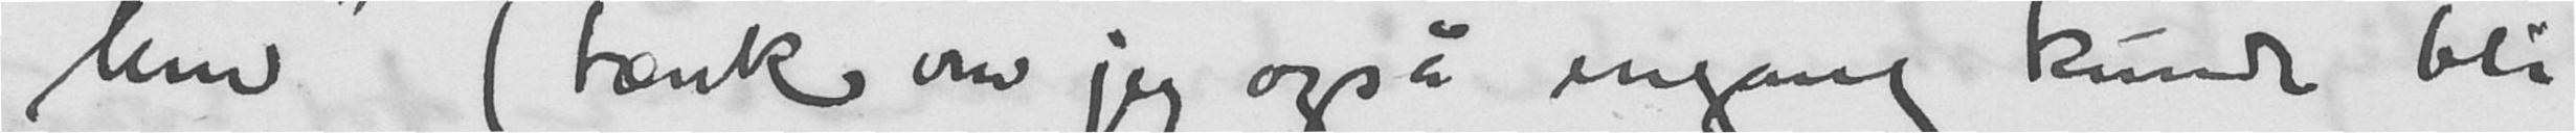lem" (tænk om jeg også engang kunde bli
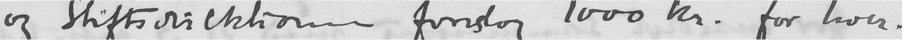og stiftsdirektionen foreslog 1000 kr. for hver.
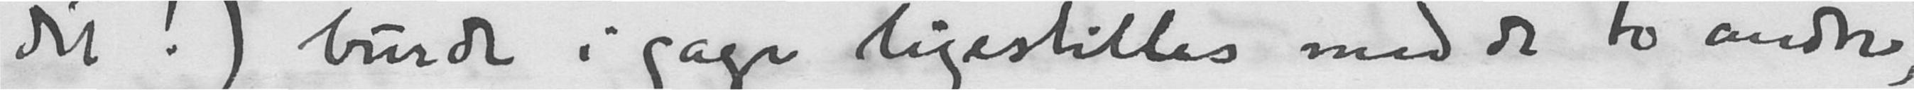det!) burde i sagen ligestilles med de to andre,
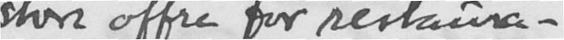store offre for restaura-
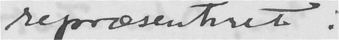repræsenteret i
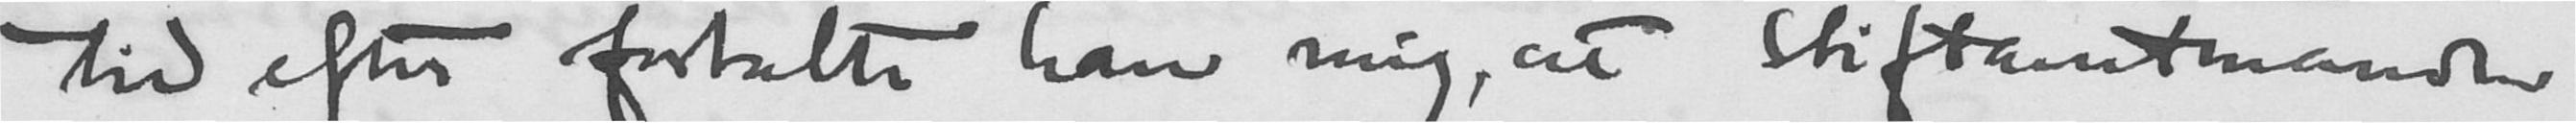tid efter fortalte han mig, at stiftamtmanden
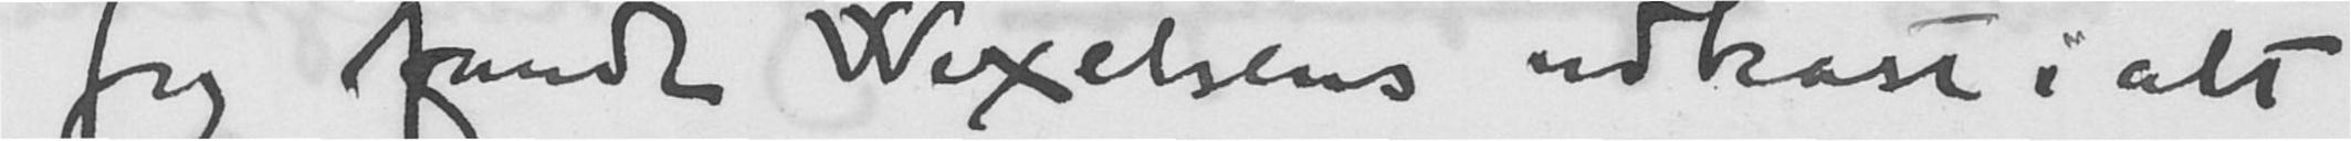Jeg fandt Wexelsens udkast i alt
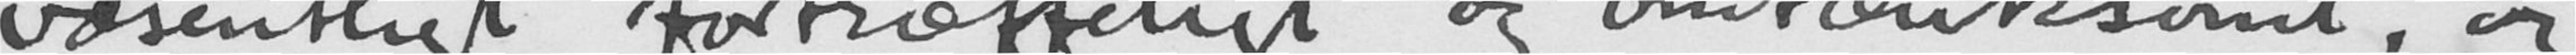væsentligt fortræffeligt og omtænktsomt, og
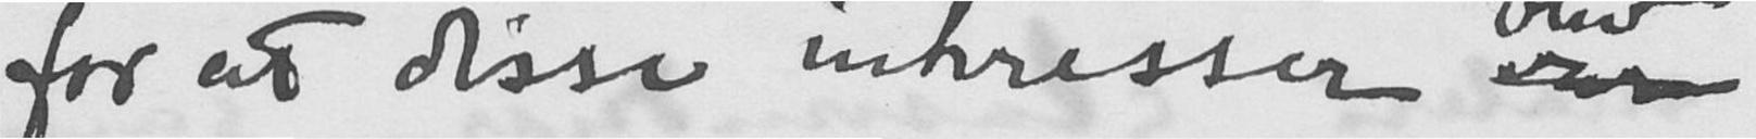for at disse interesser var
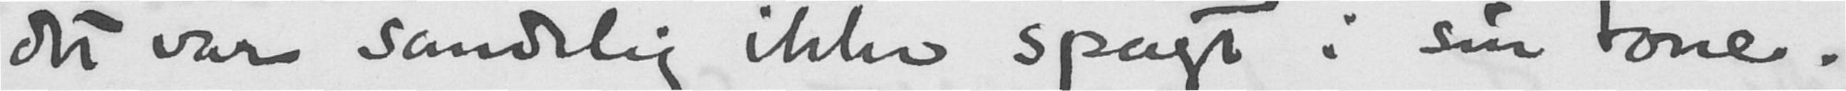det var sandelig ikke spagt i sin tone.
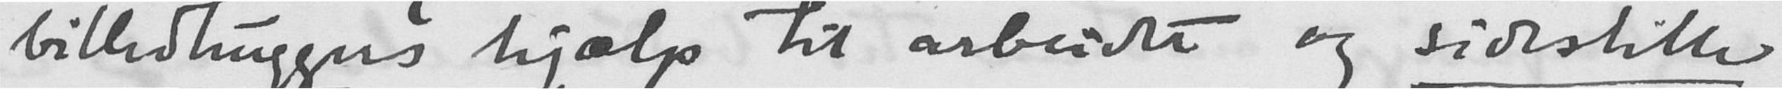billedhuggers hjælp til arbeidet og sidestille
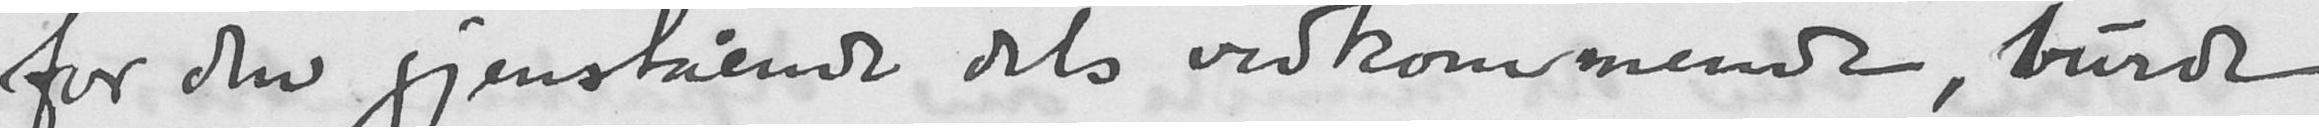for den gjenstående dels vedkommende, burde
